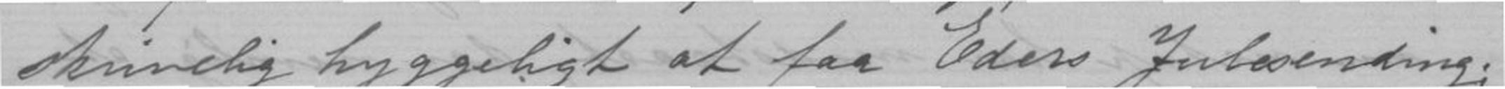skrivelig hyggeligt at faa Eders Julesending;
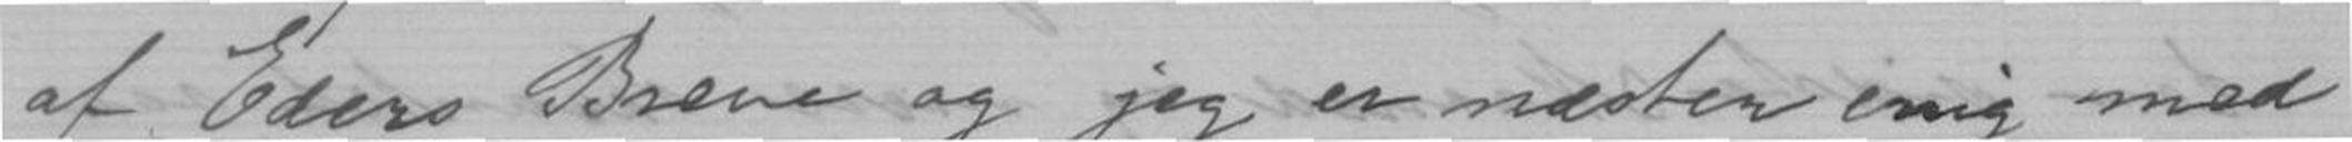af Eders Breve og jeg er næsten enig med
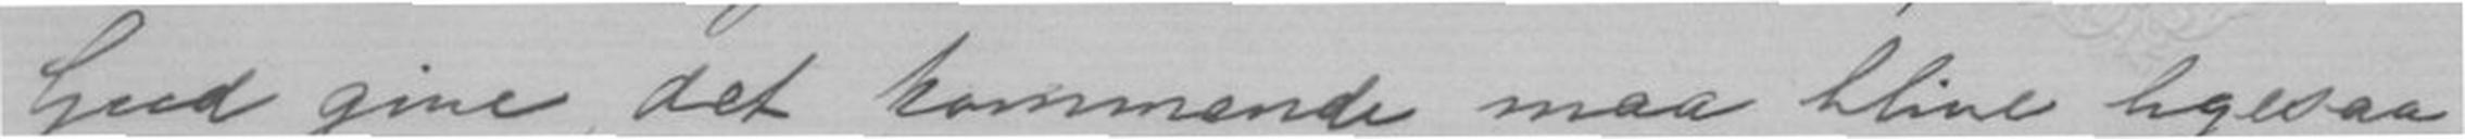Gud give, det kommende maa blive ligesaa
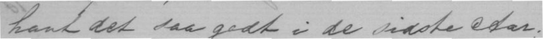havt det saa godt i det sidste Aar;
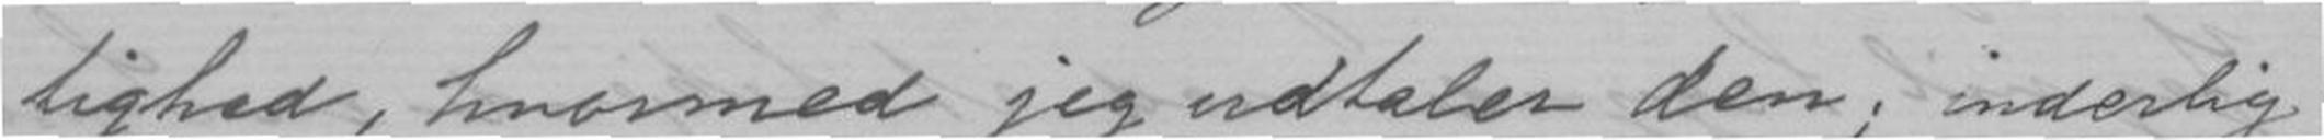tighed, hvormed jeg udtaler den; inderlig
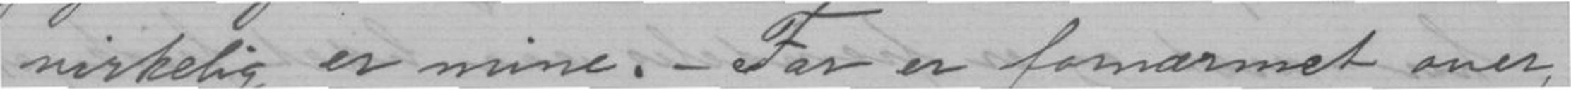virkelig er mine. - Far er fornærmet over,
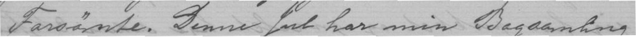Forsømte. Denne Jul har min Bogsamling
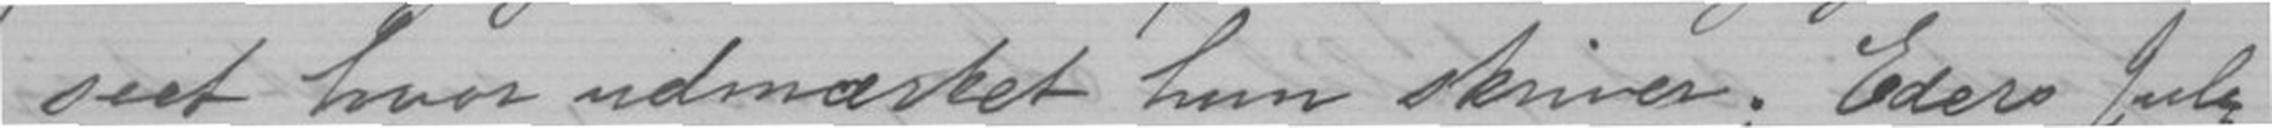seet hvor udmærket hun skriver; Eders Jule
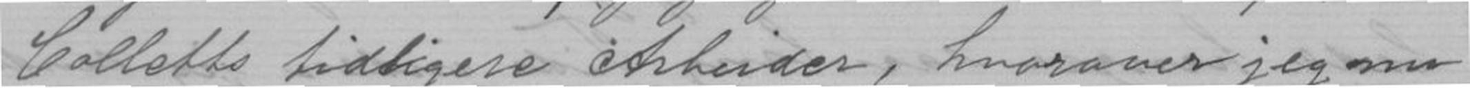Collets tidligere Arbeider, hvorover jeg nu
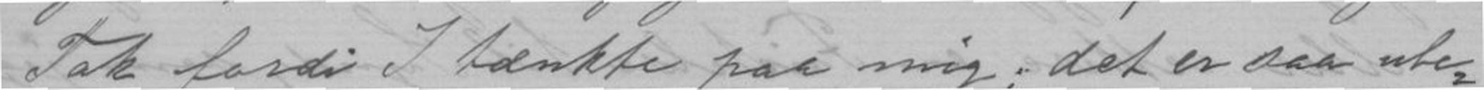Tak fordi I tænkte paa mig; det er saa ube-
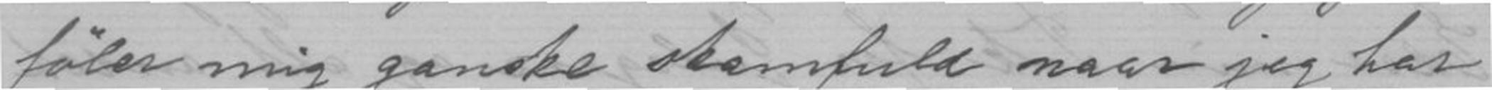føler mig ganske skamfuld naar jeg har
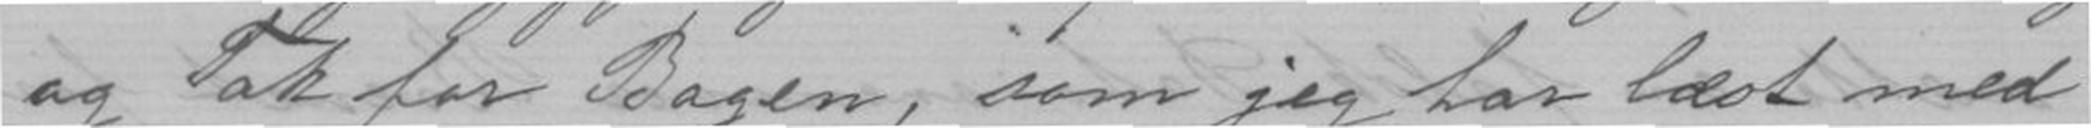og Tak for Bogen, som jeg har læst med
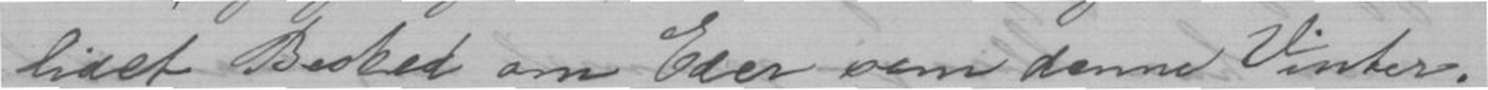lidet Besked om Eder som denne Vinter;
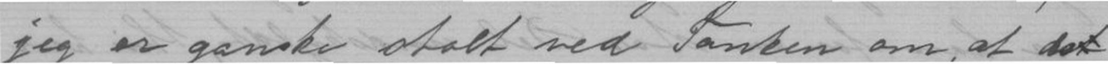jeg er ganske stolt ved Tanken om, at det
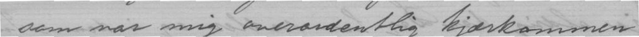som var mig overordentlig kjærkommen,
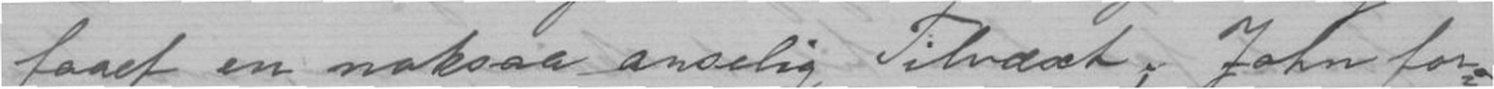faaet en noksaa anseelig Tilvæxt; John for-
